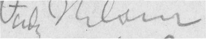Venlig Hilsen
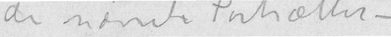de nævnte Portrætter –
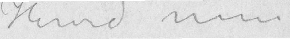Herved min
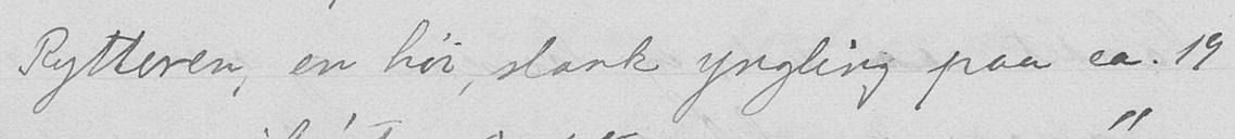Rytteren, en høi, slank yngling paa ca. 19
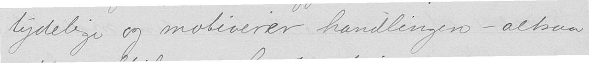tydelige og motiverer handlingen - altsaa
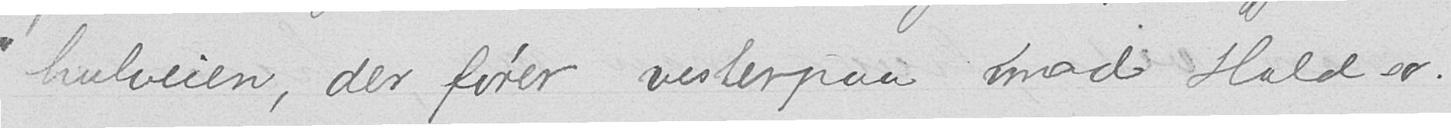hulveien, der fører vesterpaa imod Hald sø.
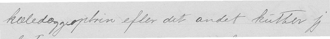kæledæggeoptrin efter det andet kutter jeg
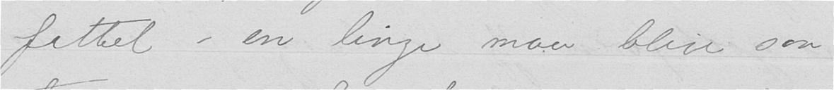fattet - en linje maa blive saa
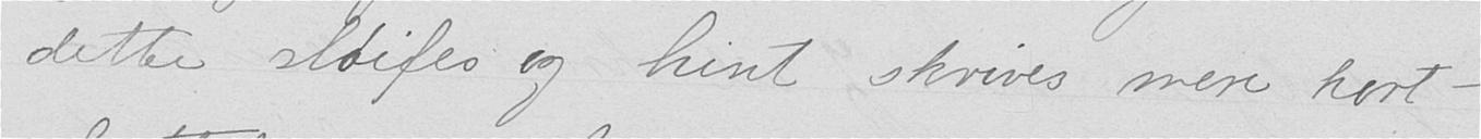dette sløifes og hint skrives mere kort-
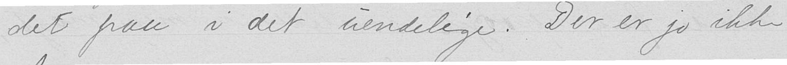det paa i det uendelige. Der er jo ikke
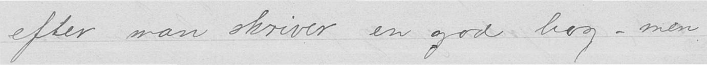efter man skriver en god bog - men
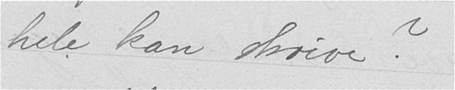hele kan skrive?
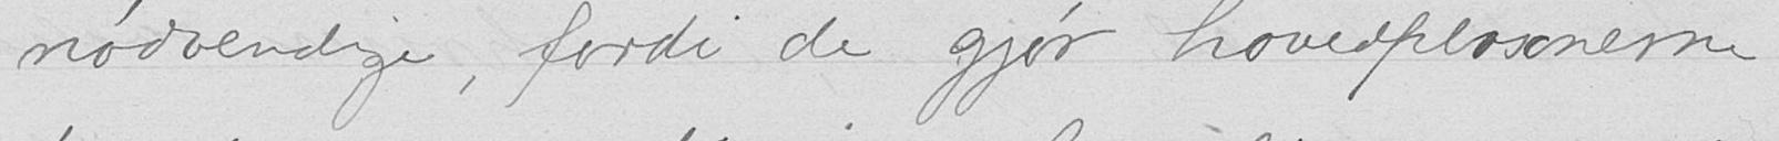nødvendige, fordi de gjør hovedpersonerne
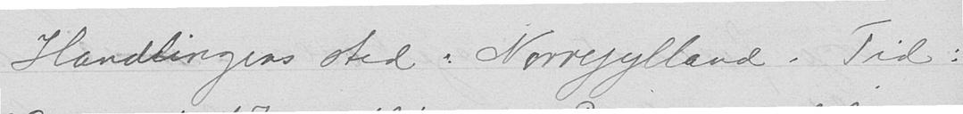Handlingens sted: Nørrejylland. Tid:
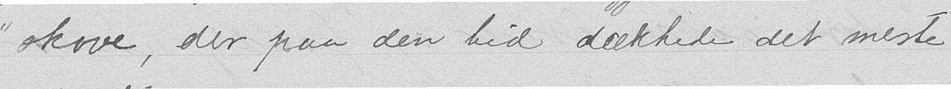skove, der på den tid dækkede det meste
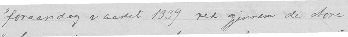"foraarsdag i aaret 1339 ret gjennem de store
